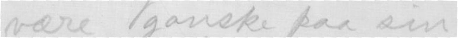være ganske paa sin
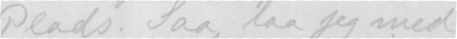Plads. Saa laa jeg med
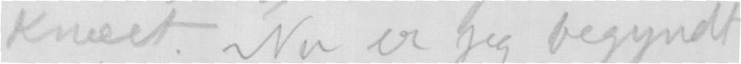Kneet. nu er jeg begyndt
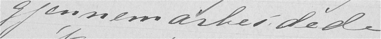gjennemarbeidede
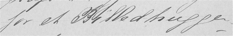for et Billedhugger-
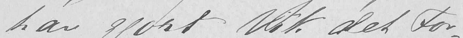har gjort Wik det For-
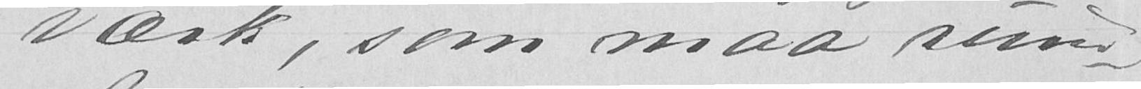værk, som maa rum-
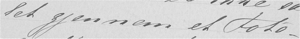let gjennem et Foto-
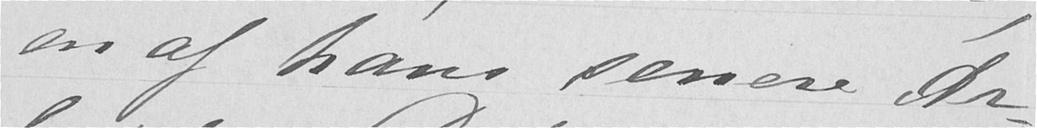en af hans senere Ar-
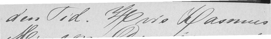den Tid. Hvis Rasmus
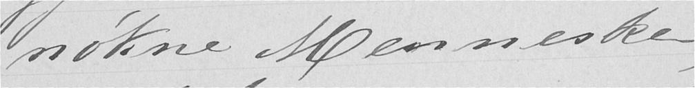nøkne Menneske,
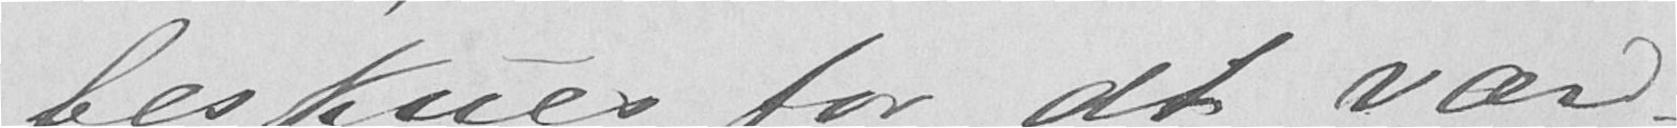beskues for at værd-
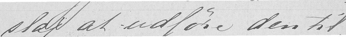slag at udføre den til
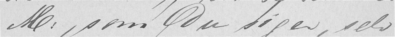M., som Du siger, selv
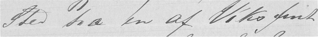Sted ha en af Viks fint
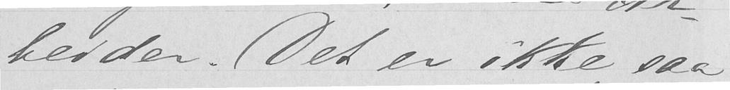beider. Det er ikke saa
